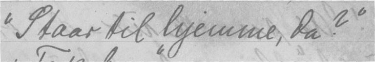"Staar til hjemme, da?"
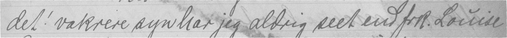det! vakrere syn har jeg aldrig seet end frk. Louise
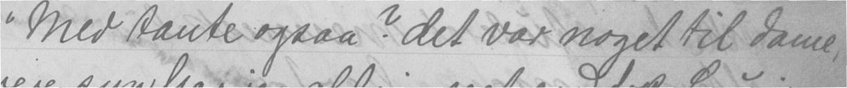"Med tante ogsaa? det var noget til dame,
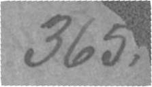365.
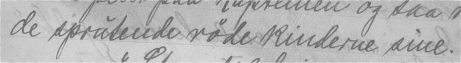de sprutende røde kinderne sine.
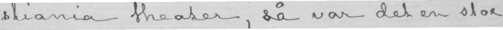stiania theater, så var det en stor
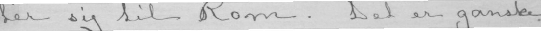ter sig til Rom. Det er ganske
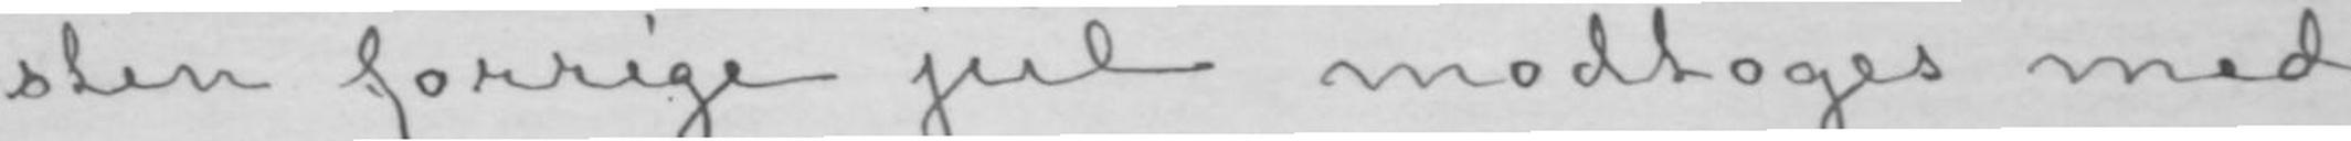sten forrige jul modtoges med
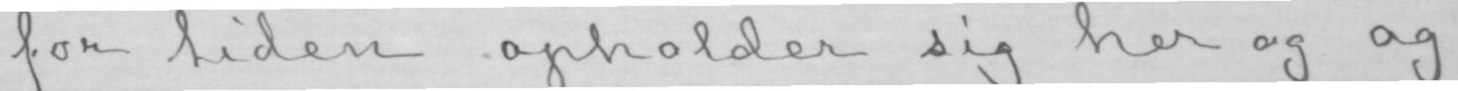for tiden opholder sig her og ag-
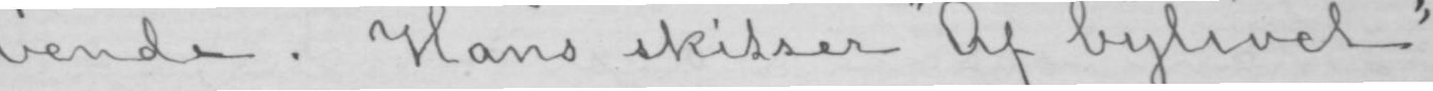vende. Hans skitser «Af bylivet»
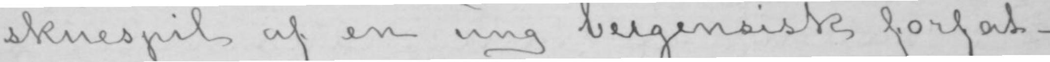skuespil af en ung bergensisk forfat-
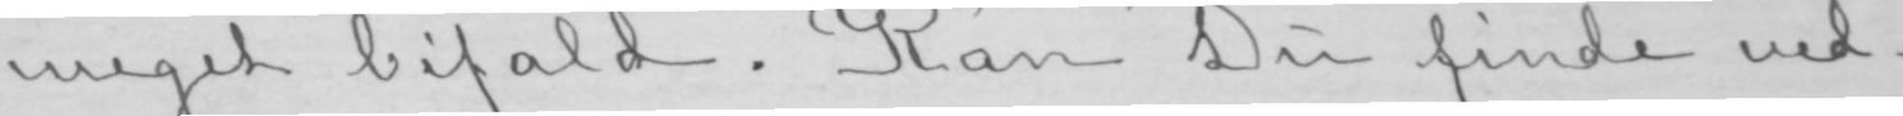meget bifald. Kan Du finde ved-
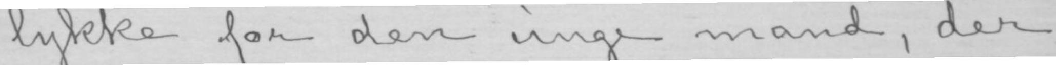lykke for den unge mand, der
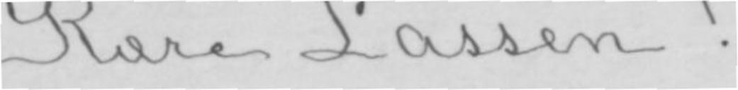Kære Lassen!
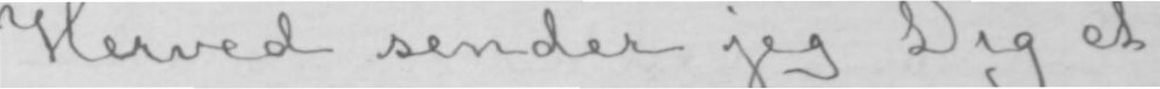Herved sender jeg Dig et
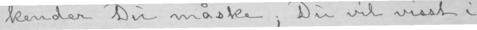kender Du måske; Du vil visst i
